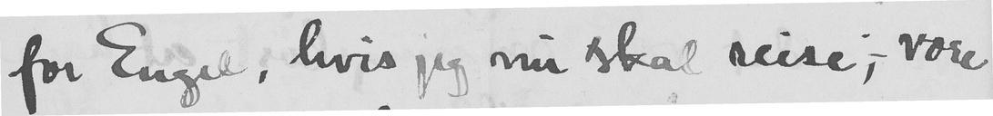for Engel, hvis jeg nu skal reise; - vore
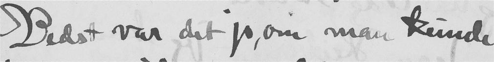Bedst var det jo, om man kunde
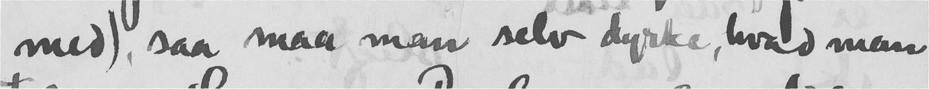med), saa maa man selv dyrke, hvad man
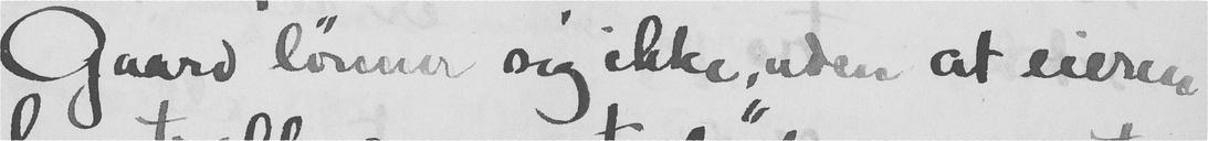Gaard lønner sig ikke, uden at eieren
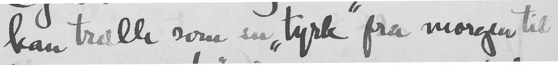kan trælle som en "tyrk" fra morgen til
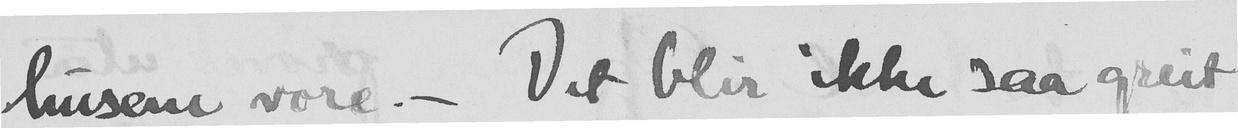husene vore. - Det blir ikke saa greit
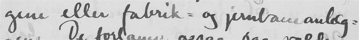gene eller fabrik- og jernbaneanlæg-
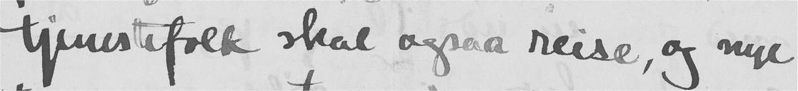tjenestefolk skal ogsaa reise, og nye
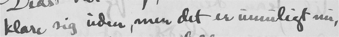klare sig uden, men det er umuligt nu,
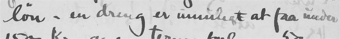løn - en dreng er umuligt at faa under
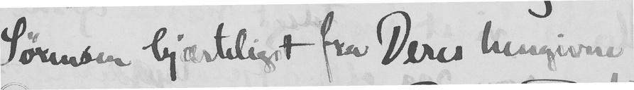Sørensen hjærteligst fra Deres hengivne
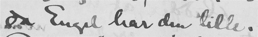da Engel har den lille.
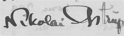Nikolai Astrup
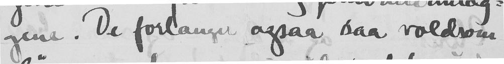gene. De forlanger ogsaa saa voldsom
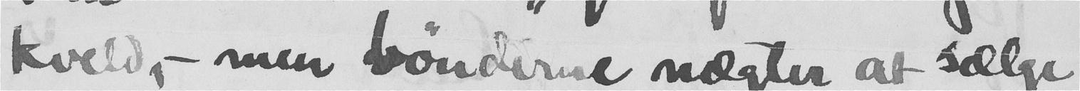kveld, - men bønderne nægter at sælge
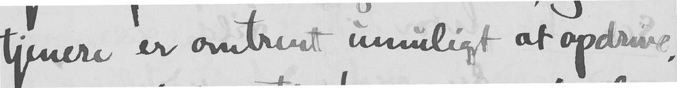tjenere er omtrent umuligt at opdrive,
